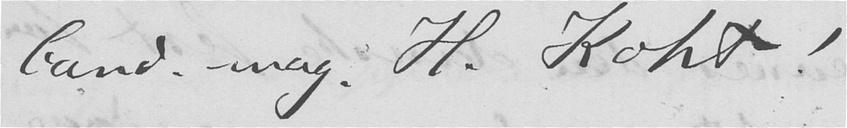Cand. mag. H. Koht!
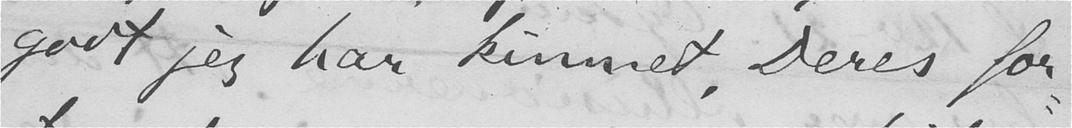godt jeg har kunnet, Deres for-
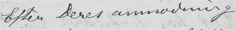Efter Deres anmodning
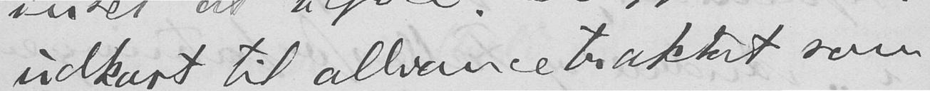udkast til alliancetraktat som
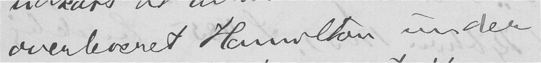overleveret Hamilton under
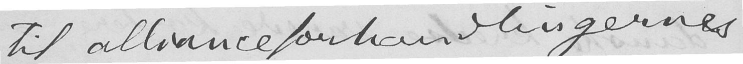til allianceforhandlingernes
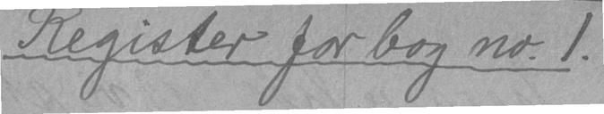Register for bog. no. 1.
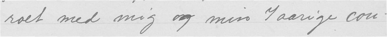roet med mig og min 7aarige cou-
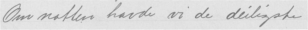Om natten havde vi de deiligste
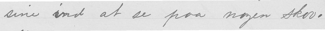sine ind at se paa nogen skov.
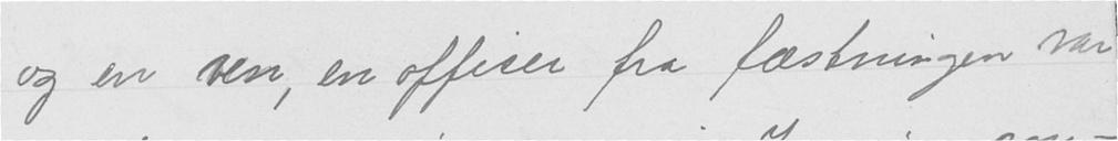og en ven, en officer fra fæstningen var
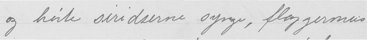og hørte siridserne synge, flaggermus
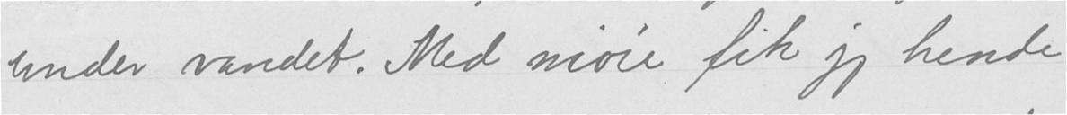under vandet. Med møie fik jeg hende
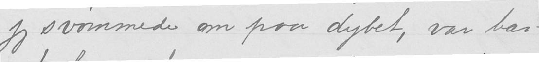jeg svømmede om paa dybet, var bar-
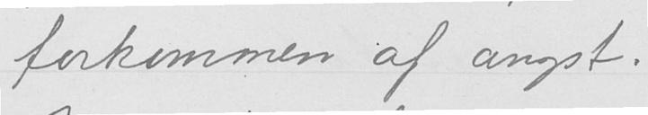forkommen af angst.
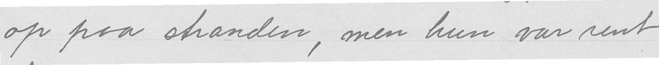op paa stranden, men hun var rent
